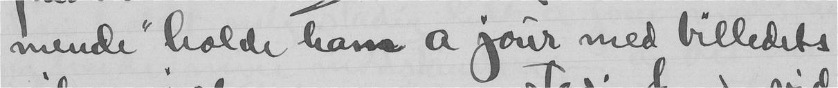mende" holde ham a jour med billedets
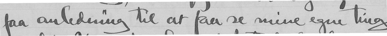faa anledning til at faa se mine egne ting
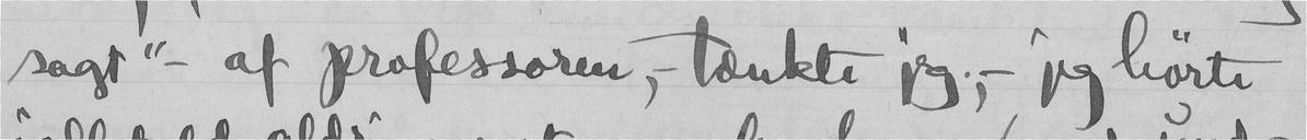sagt" - af professoren, - tænkte jeg, - jeg hørte
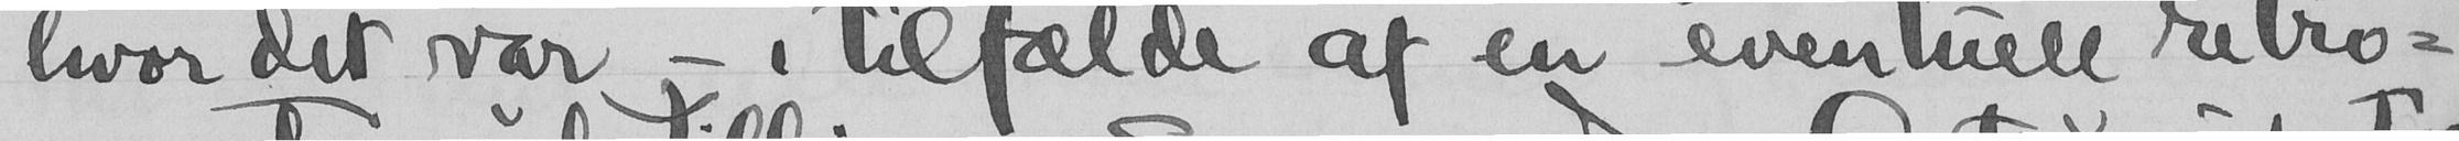hvor det var - i tilfælde af en eventuell retro-
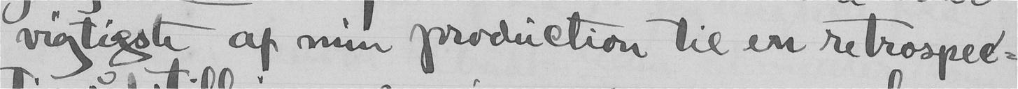vigtigste af min production til en retrospec-
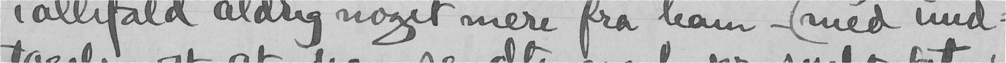iallefald aldrig noget mere fra ham - (med und-
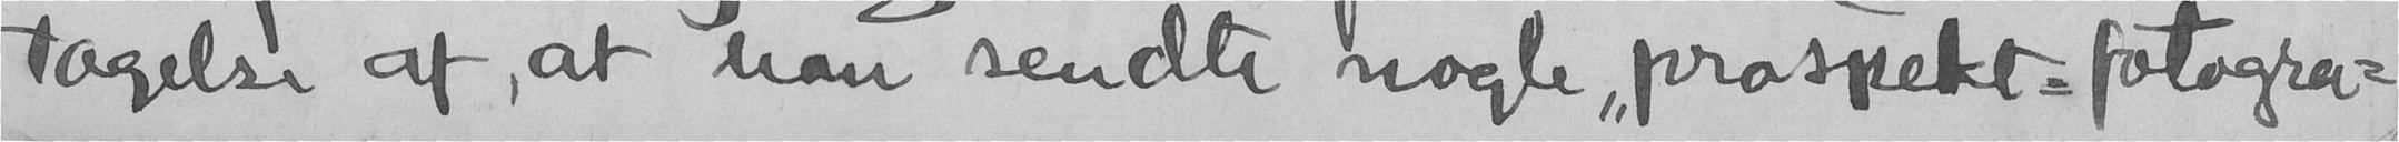tagelse af, at han sendte nogle "prospekt-fotogra-
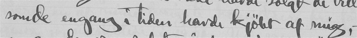som de engang i tiden havde kjøbt af mig,-
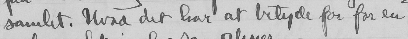samlet, Hvad det har at betyde for for en
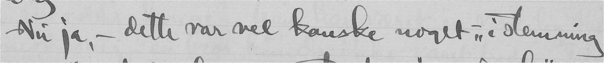Nu ja, - dette var vel kanske noget - "i stemning
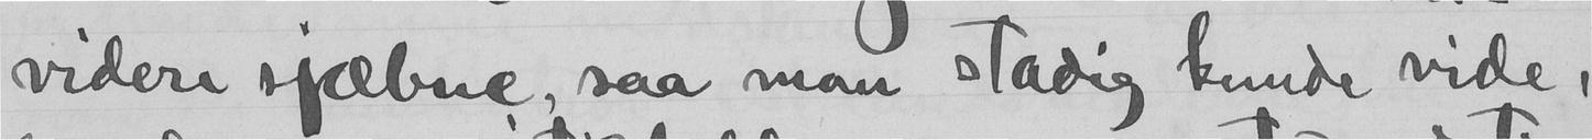videre sjæbne, saa man stadig kunde vide
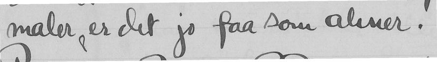maler "er det jo faa som almer.
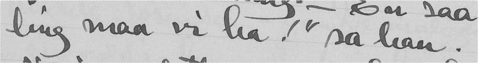ling maa vi ha!" sa han.
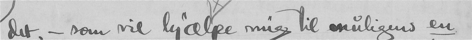det - som vil hjælpe mig til mnuligens en
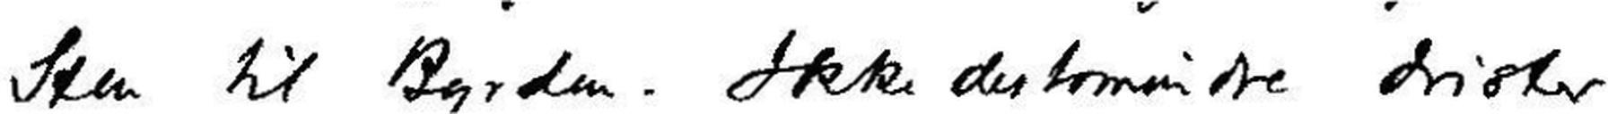Sten til Byrden. Ikke destomindre drister
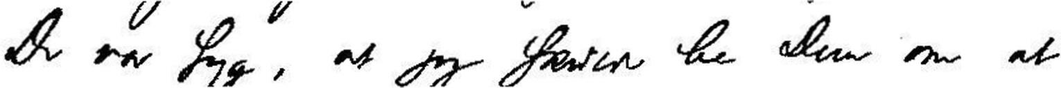De var Syg, at jeg skulde be Dem om at
